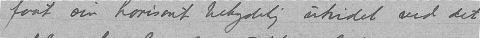faat sin horisont betydelig utvidet ved det
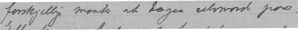forskjellige maater at begaa selvmord paa.
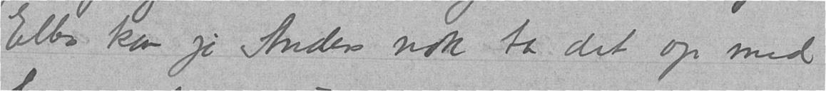Ellers kan jo Anders nok ta det op med
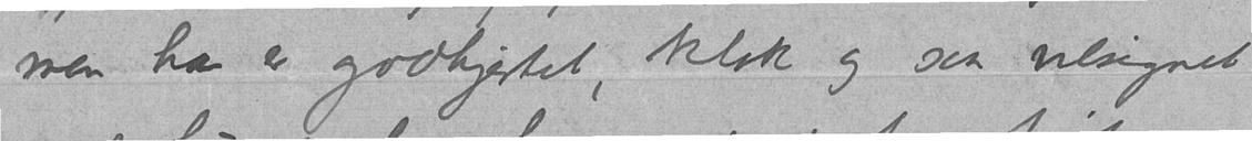men han er godhjertet, klok og saa velsignet
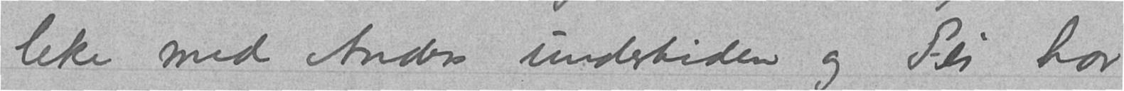leke med Anders undertiden og Pei har
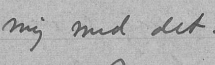mig med det.
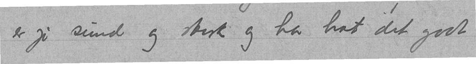er jo sund og sterk og har hat det godt
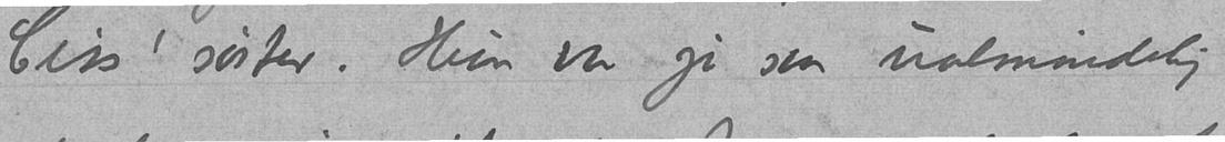Ciss' søster. Hun var jo saa ualmindelig
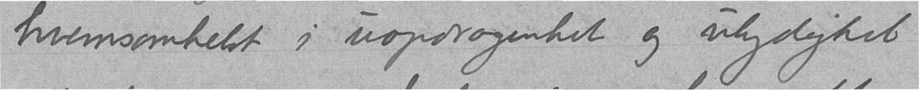hvemsomhelst i uopdragenhet og ulydighet
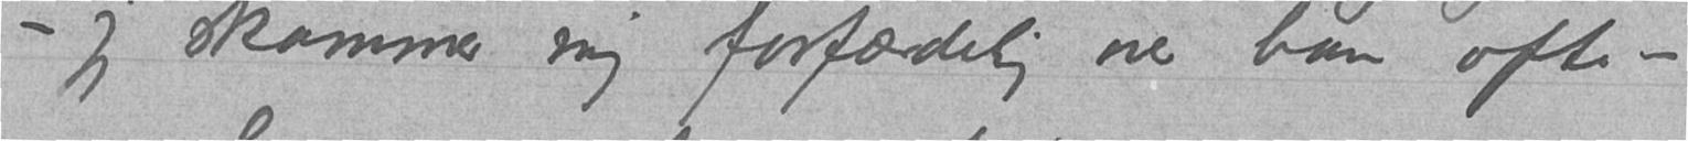- jeg skammer mig forfærdelig over ham ofte -
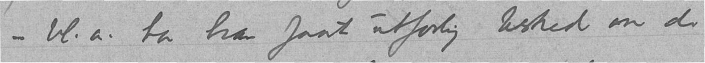- bl.a. har han faat utførlig besked om de
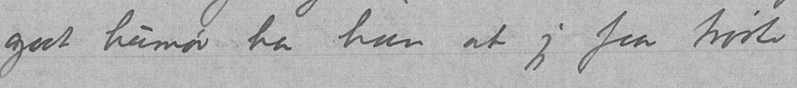godt humør har han at jeg faar trøste
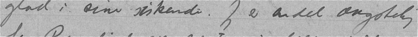glad i sine søskende. Jeg er endel ængstelig
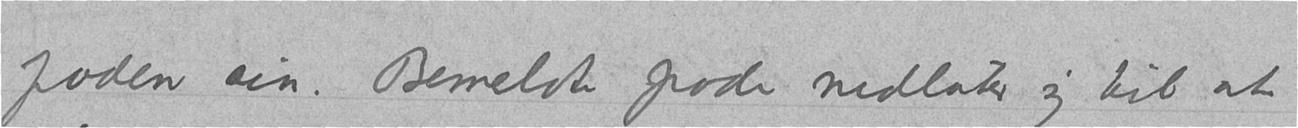poden sin. Bemeldte pode nedlater sig til at
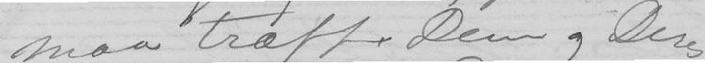maa træffe Dem og Deres
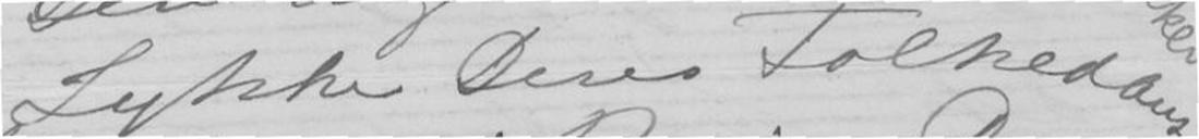Lykke Deres Folkedanse
